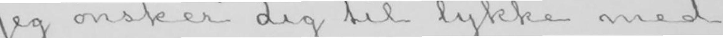Jeg ønsker dig til lykke med
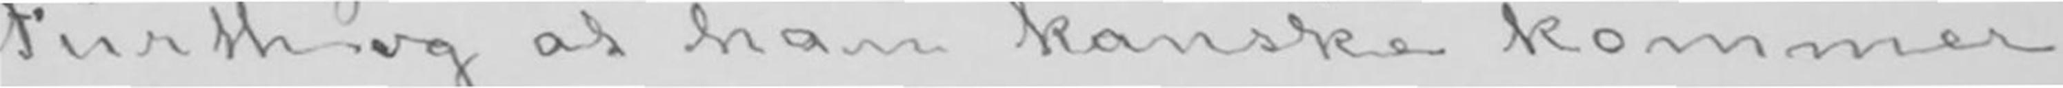Fürth og at han kanske kommer
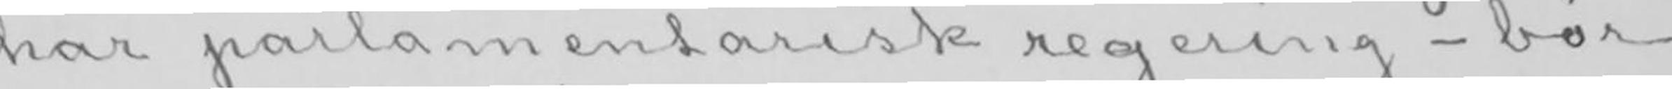har parlamentarisk regering- bør
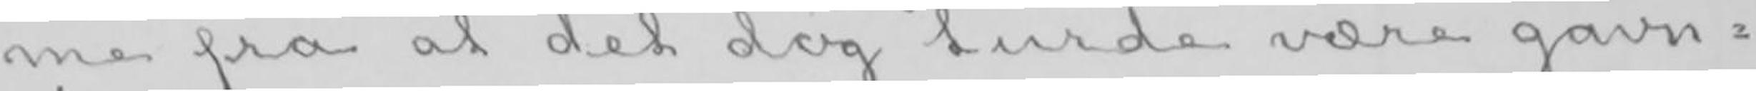me fra at det dog turde være gavn-
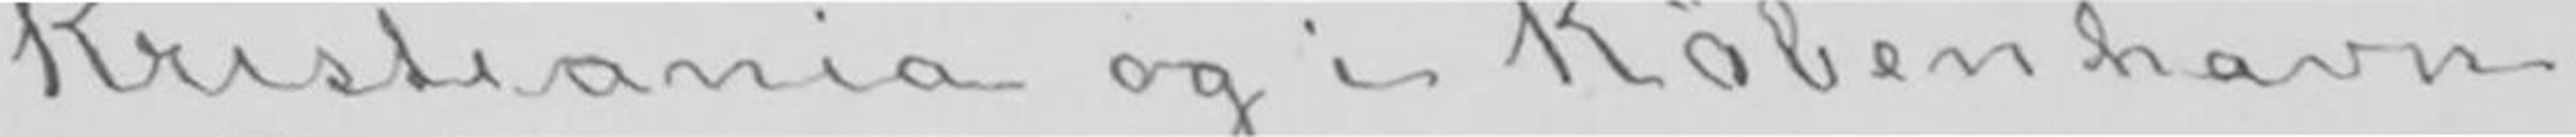Kristiania og i København.
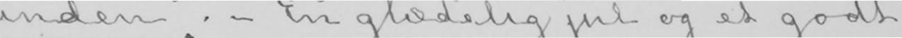inden.- En glædelig jul og et godt
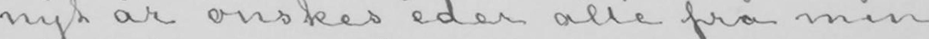nyt år ønskes eder alle fra min
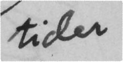tider
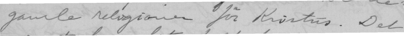gamle religioner før Kristus. Det
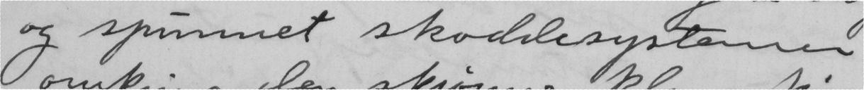og spunnet skoddesystemer
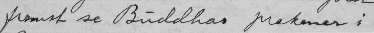fremst se Buddhas prekener i
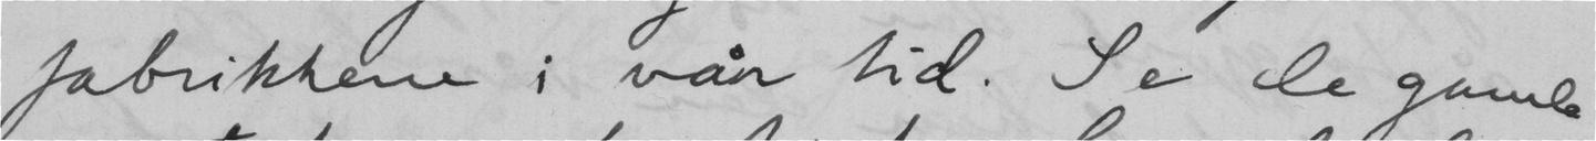fabrikkene i vår tid. Se de gamle
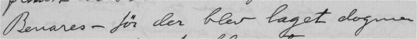Benares - før der blev laget dogmer
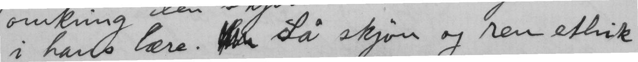i hans lære.  Så skjøn og ren ethik
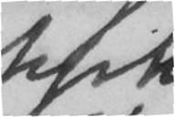kjok
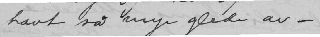havt så mye glede av -
